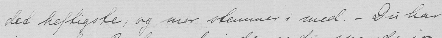det heftigste; og mor stemmer i med. – Du har
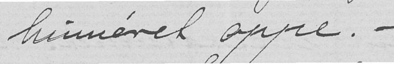humøret oppe. –
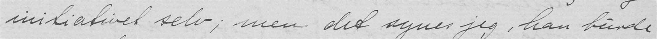initiativet selv; men det synes jeg, han burde
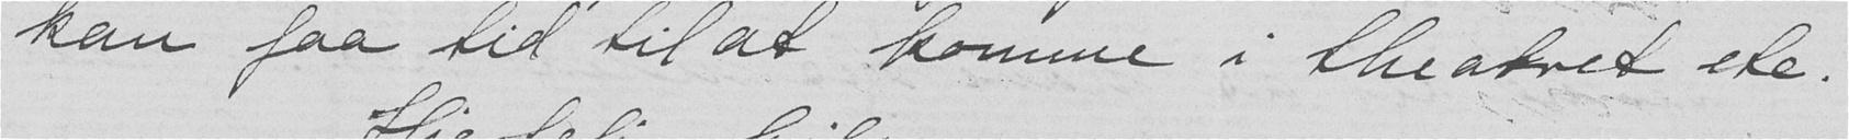kan faa tid til at komme i theatret etc.
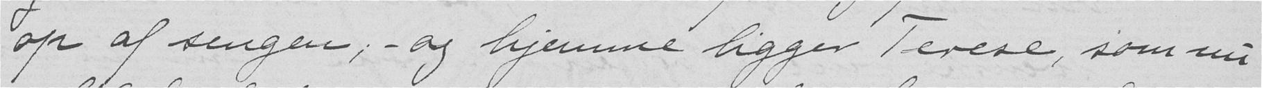op af sengen; – og hjemme ligger Terese, som nu
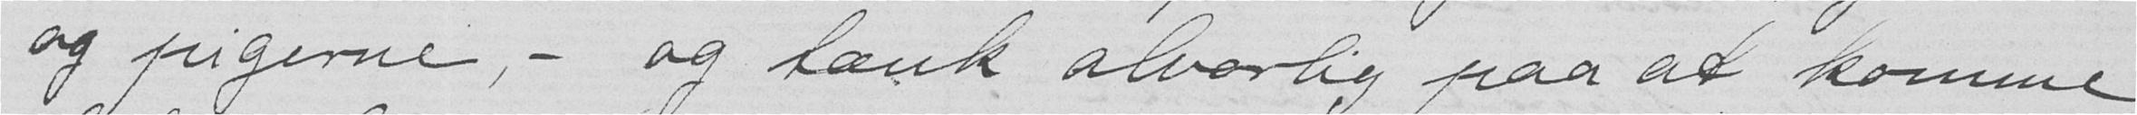og pigerne, – og tænk alvorlig paa at komme
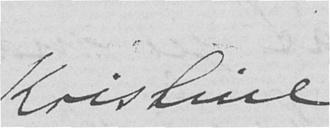Kristine
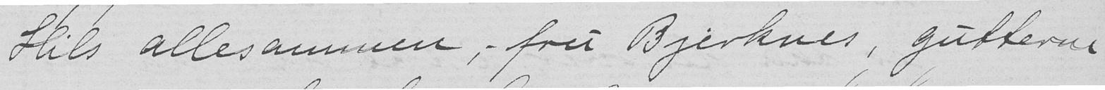Hils allesammen, – fru Bjerknes, gutterne
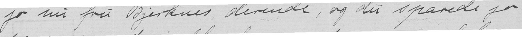jo nu fru Bjerknes derinde, og du sparede jo
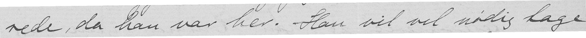rede, da han var her. Han vil vel nødig tage
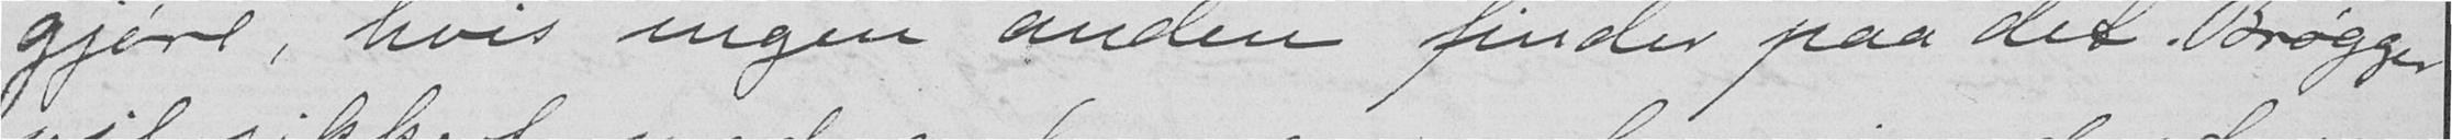gjøre, hvis ingen anden finder paa det. Brøgger
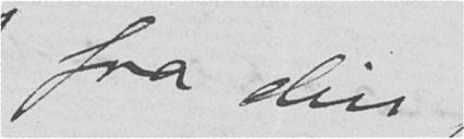fra din
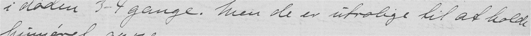i døden 3-4 gange. Men de er utrolige til at holde
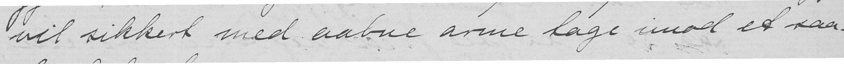vil sikkert med aabne arme tage imod et saa-
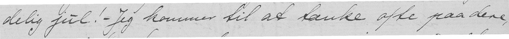delig jul! – Jeg kommer til at tænke ofte paa dere,
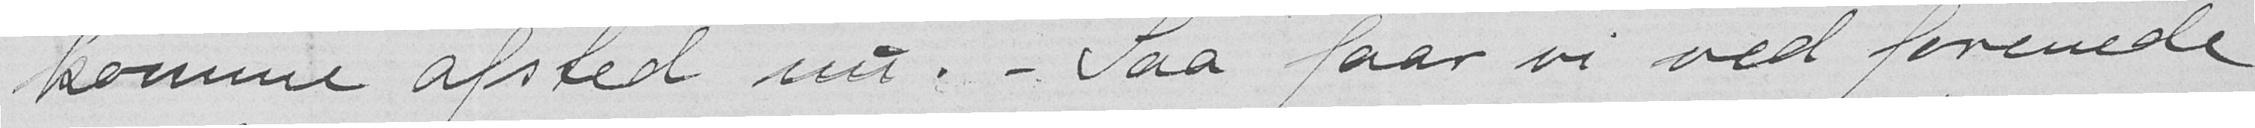komme afsted nu. – Saa faar vi ved forenede
